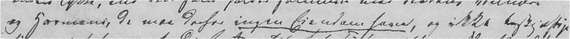og Harmoni, de maa derfor ingen Eiendom have, og ikke beskjæftige
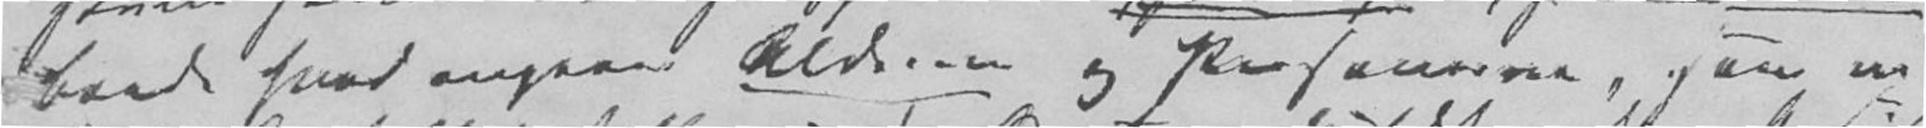baade hvad angaaer Alderen Personerne, som m.
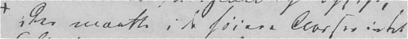Der maatte i de høiere Classer intet
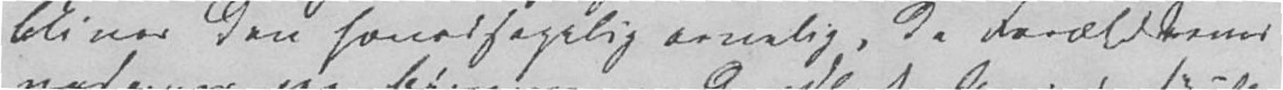bliver den hovedsagelig arvelig, da Forældrenes
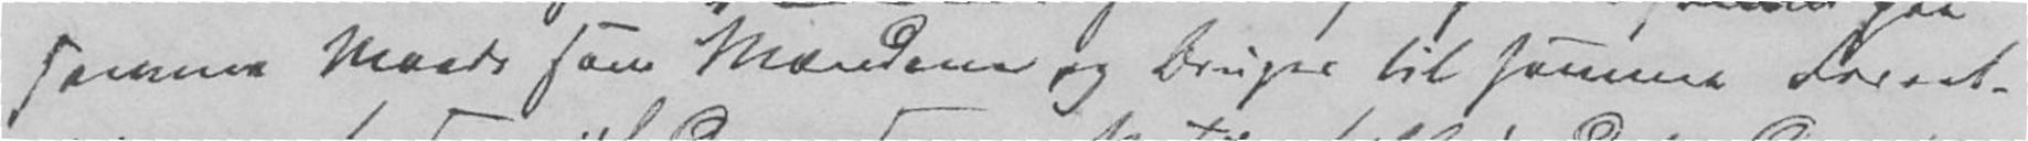samme Maade som Mændene og bruges til samme Forret-
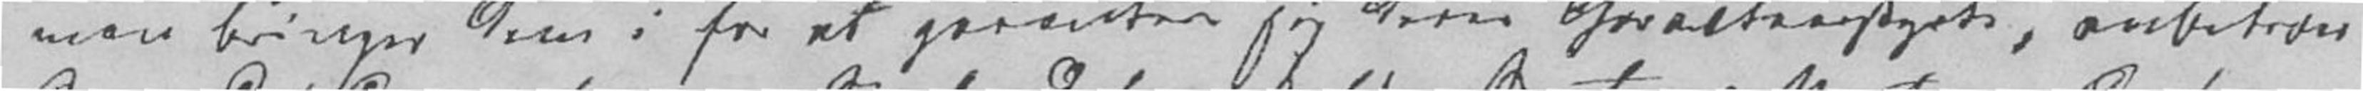man bringer dem i for at garantere sig deres Characteerstyrke, anbetroes
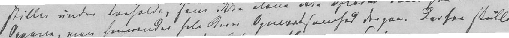Opgave, men henvender hele deres Opmærksomhed derpaa. Derfor skulle
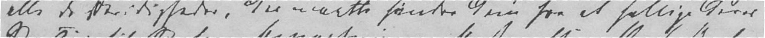alle de Stridigheder, der maatte hindre dem fra at hellige deres
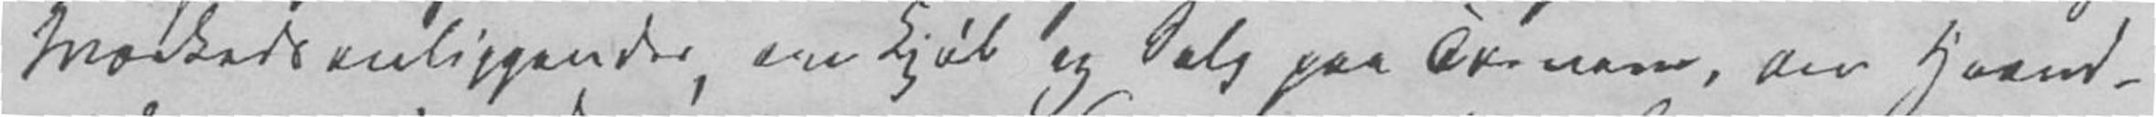Markedsanliggender, om Kjøb og Salg paa Torvene, om Haand-
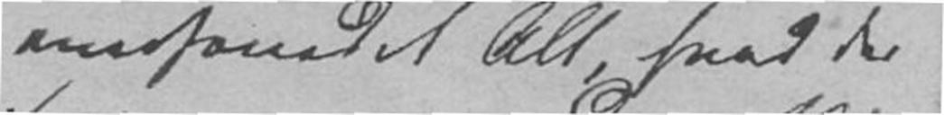overhovedet Alt, hvad der
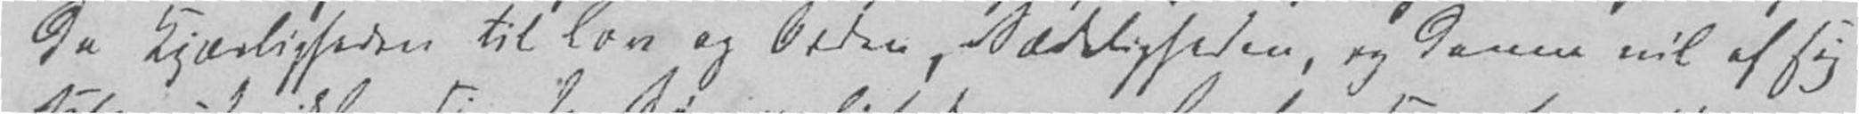da Kjærligheden til Lov og Orden, Sædeligheden, og denne vil af sig
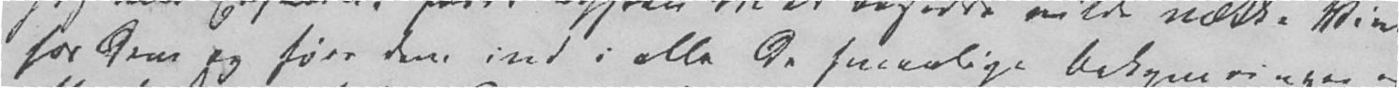hos dem og føre dem ind i alle de smaalige Bekymringer og
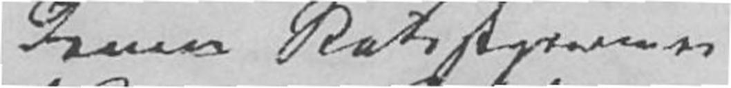Denne Statsstyrernes
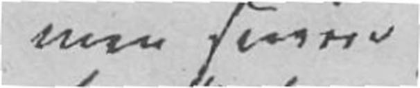men senere
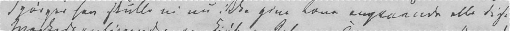spørger han skulle vi nu ikke give Love angaaende alle disse
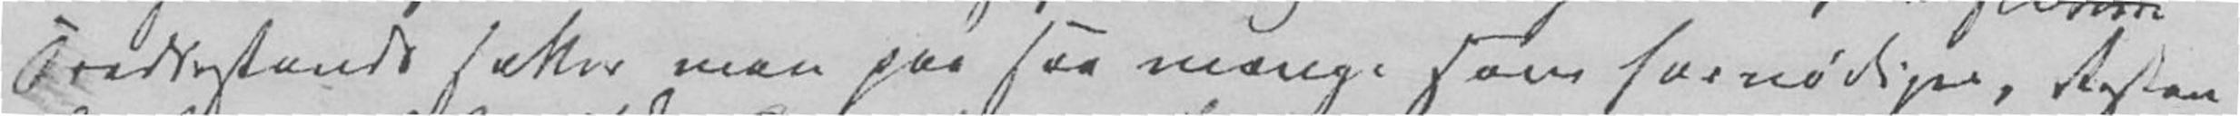Trediestands sætter man paa saa mange som fornødigen, Resten
